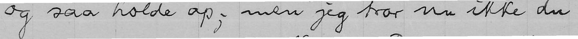og saa holde op; men jeg tror nu ikke du
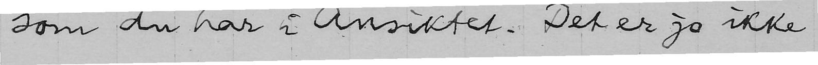som du har i Ansiktet. Det er jo ikke
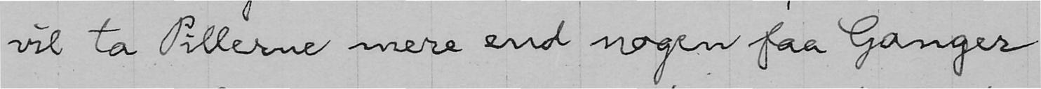vil ta Pillerne mere end nogen faa Ganger
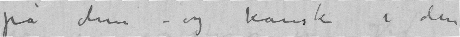på dem – og kanske i den
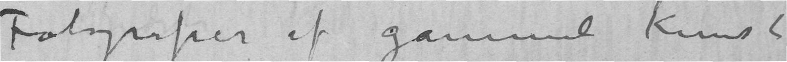Fotografier af gammel Kunst
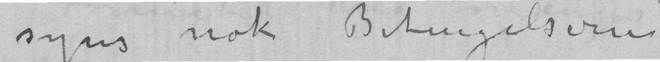synes nok Betingelserne
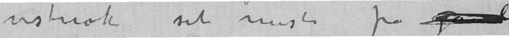vistnok set mest på gamle
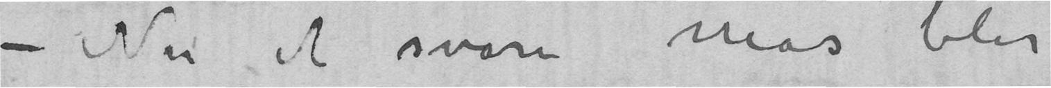–	Nu et svare Mas blir
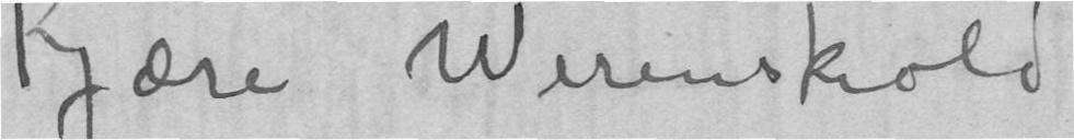Kjære Werenskiold
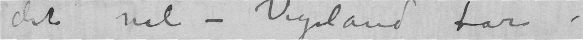det vel – Vigeland har
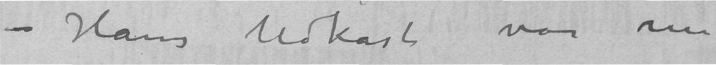–	Hans Udkast var nu
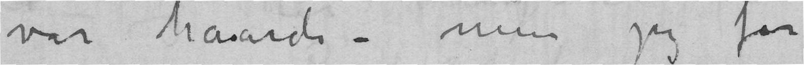var haarde – men jeg får
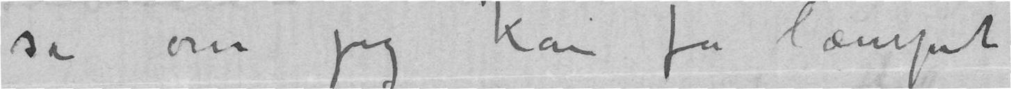se om jeg kan få læmpet
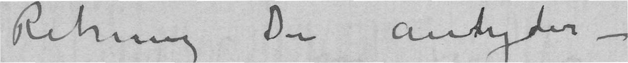Retning De antyder –
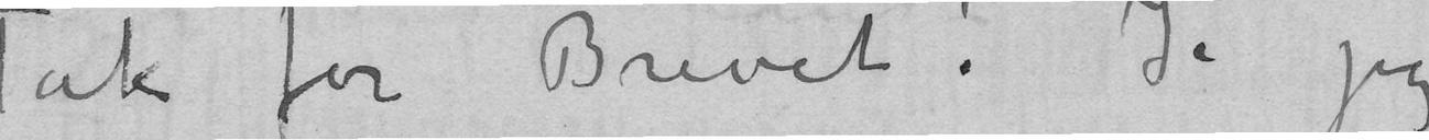Tak for Brevet! Ja jeg
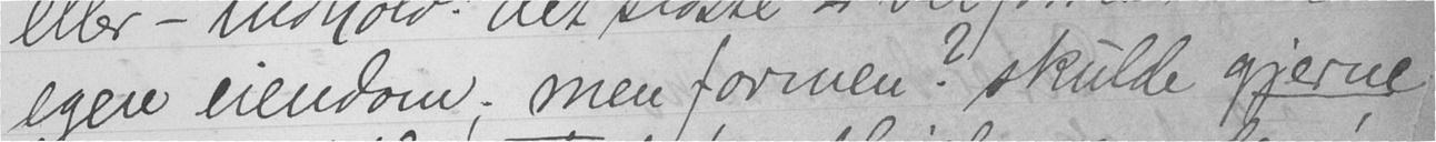egen eiendom; men formen? skulde gjerne
